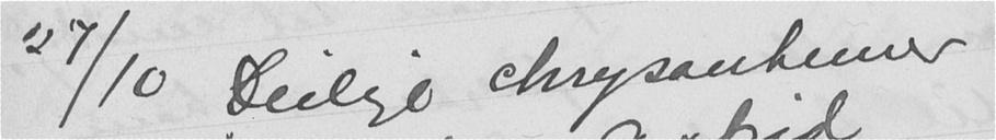27/10 Deilige chrysantemer
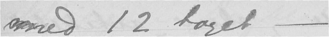med 12 toget -
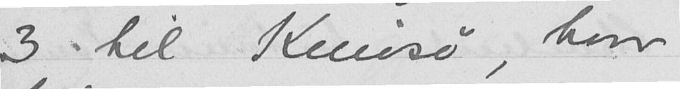3 til Knivsø, hvor
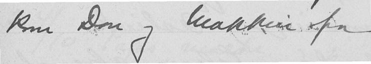kom Don og Makken fra
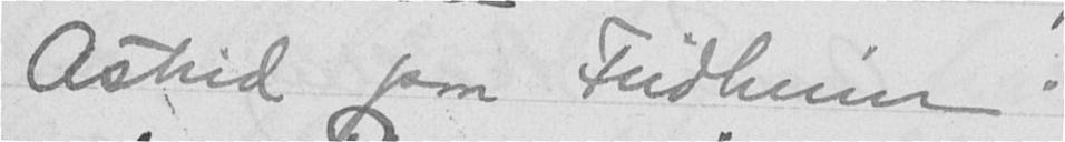Astrid paa Fridheim.
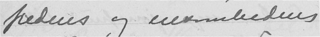fredens og ensomhedens
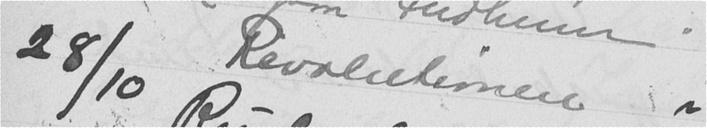28/10 Revolutionen i
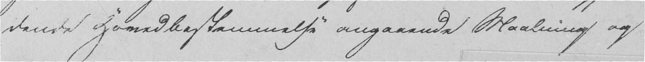dende Hovedbestemmelse angaaende Maalning og
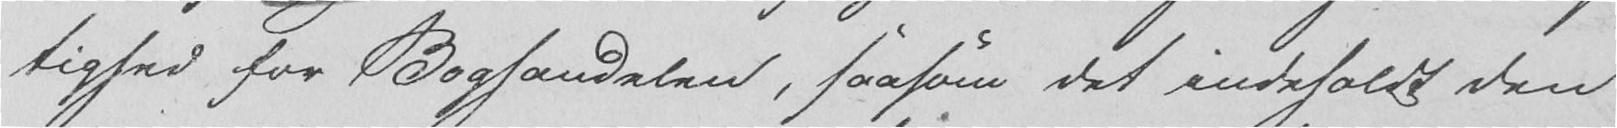tighed for Boghandelen, saasom det indeholdt den
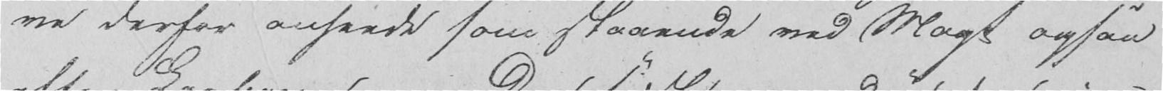ve derfor anseede som staaende ved Magt ogsaa
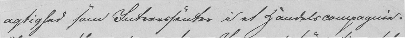agtighed som Interessenter i et Handelscompagnie.
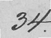34.
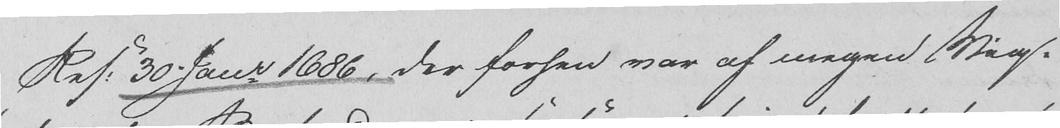Res. 30. Janr 1686, der forhen var af megen Vig-
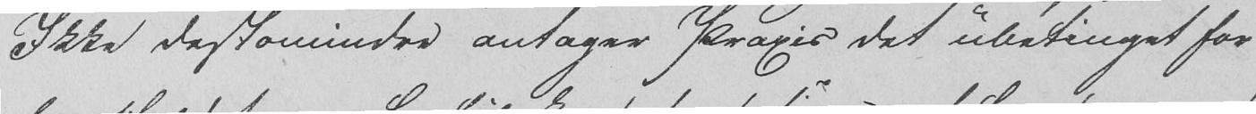Ikke destomindre antager Praxis det ubetinget for
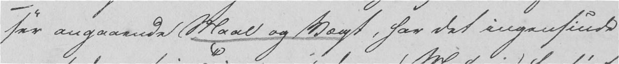ser angaaende Maal og Vægt, har det ingensinde
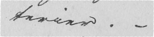terier. -
